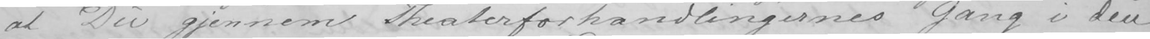at Du gjennem Theaterforhandlingernes Gang i den
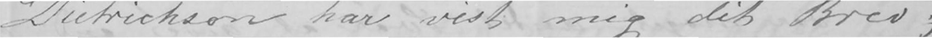Dietrichson har vist mig dit Brev;
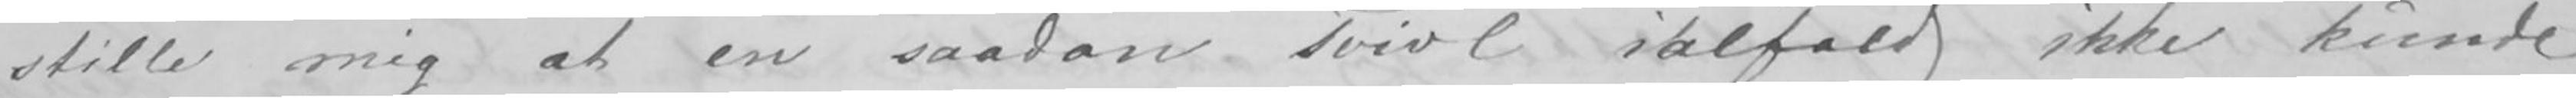stille mig at en saadan Tvivl ialfald ikke kunde
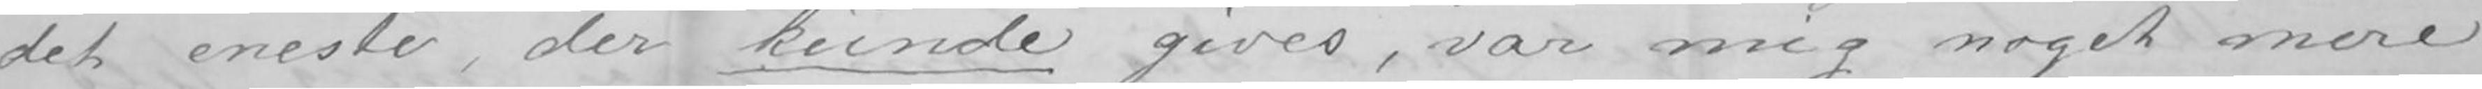det eneste, der kunde gives, var mig noget mere
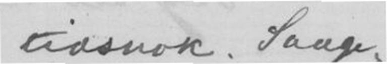tidsnok. Sange-
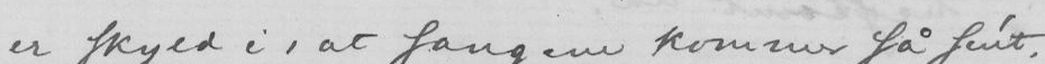er skyld i, at sangene kommer så sent.
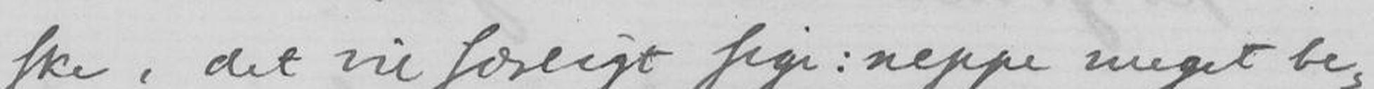ske, det vil særligt sige: neppe meget be-
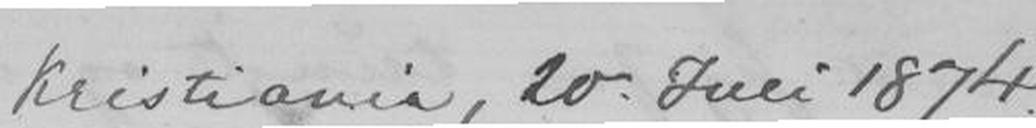Kristiania, 20 Juli 1874.
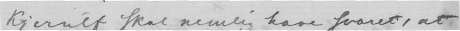Kjerulf skal nemlig have svaret, at
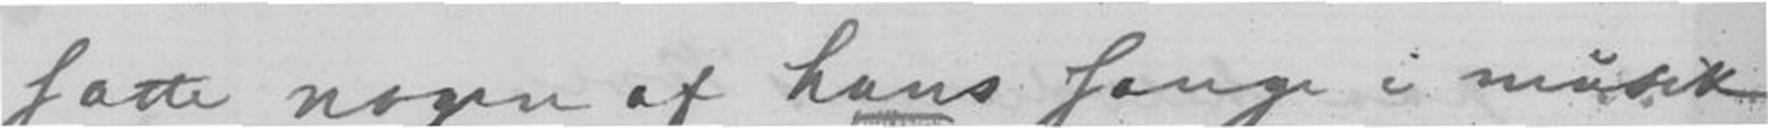satte nogen af hans sange i musik.
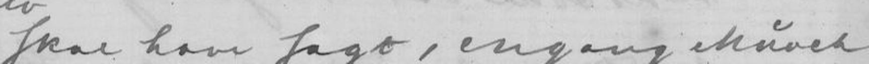skal have sagt, engang Munch
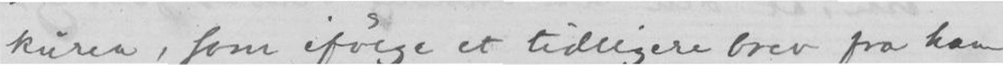kuren, som ifølge et tidligere brev fra han
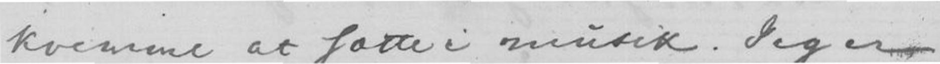kvemme at sætte i musik. Jeg er
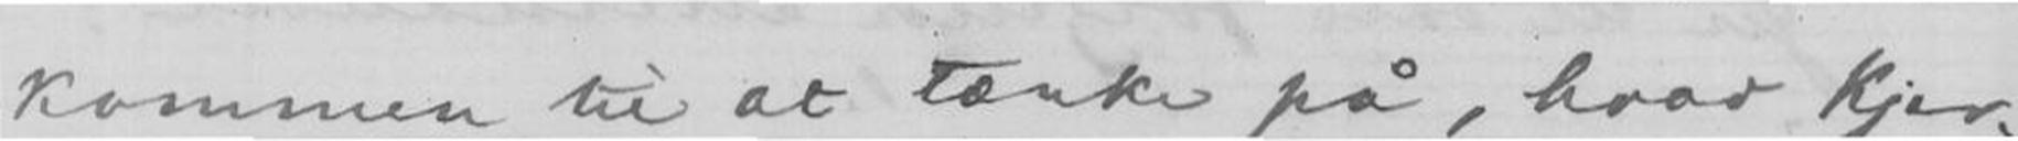kommen til at tænke på, hvad Kjer-
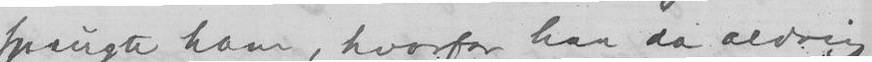spurgte ham, hvorfor han da aldrig
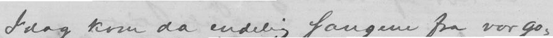I dag kom da endelig Sangene fra vor go-
